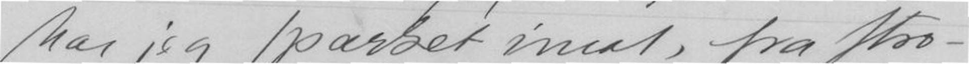har jeg sparket imot, fra stro-
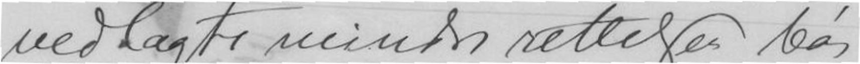vedlagte mindre rettelser bør
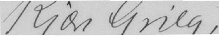Kjære Grieg,
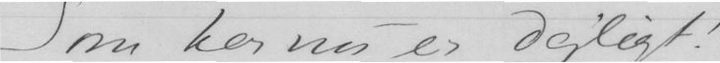Som her nu er dejligt!
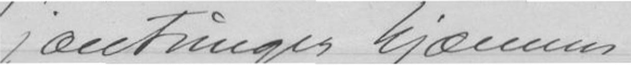jæntunger hjæmme
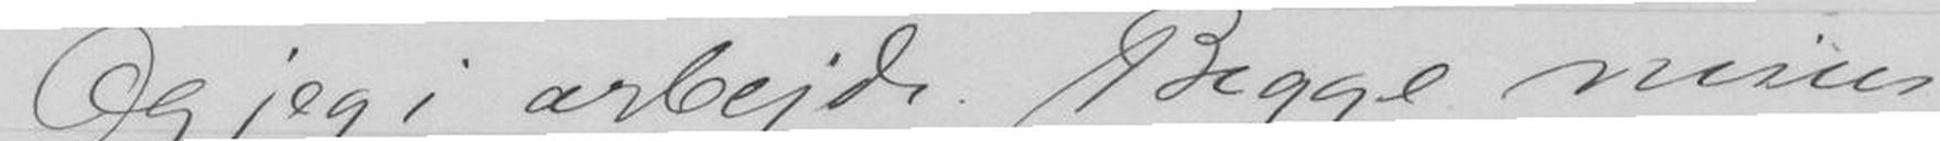Og jeg i arbejde. Begge mine
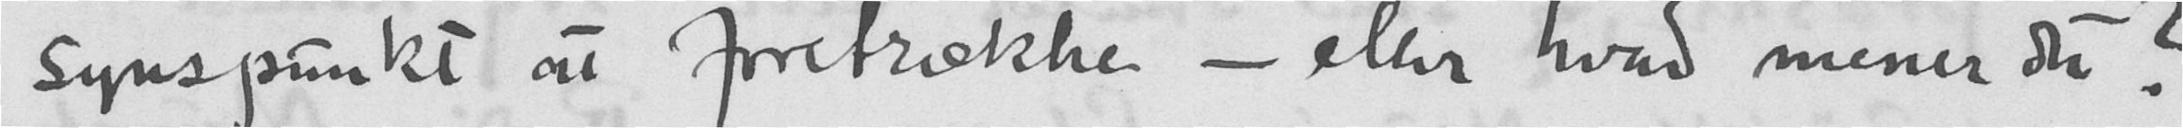synspunkt at foretrække - eller hvad mener du?
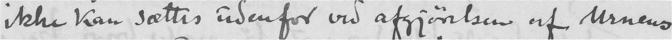ikke kan sættes udenfor ved afgjørelsen af Urnens
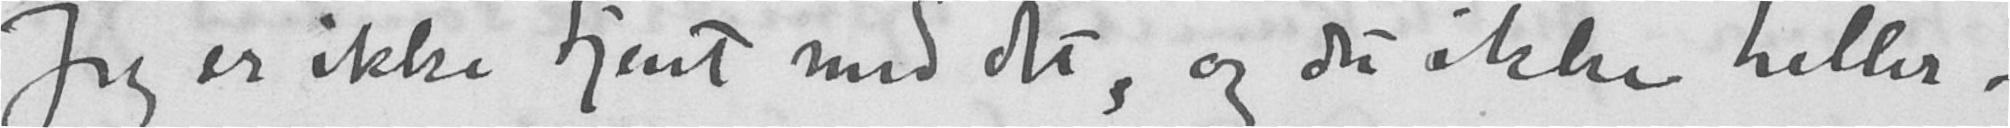Jeg er ikke tjent med det, og du ikke heller.
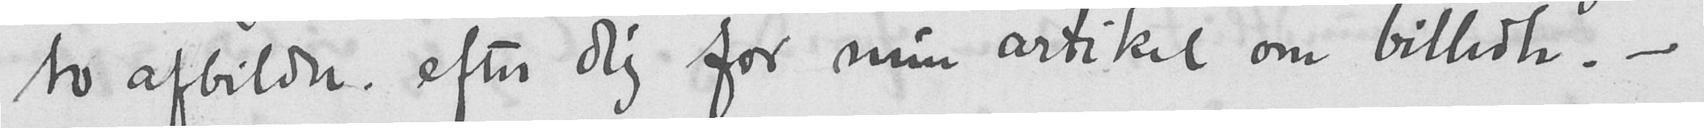to afbildn. eften dig for min artikel om billedh. -
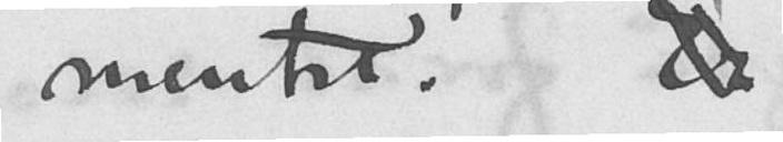mentet! Er
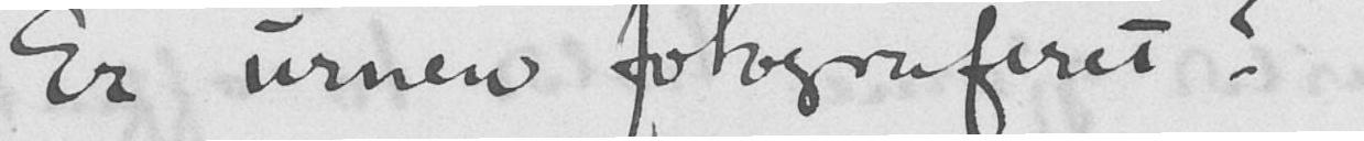Er urnen fotograferet?
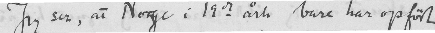- Jeg ser, at "Norge i 19de årh" bare har opført
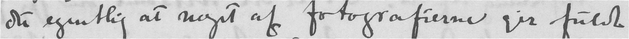du egentlig at noget af fotografierne gir fuldt
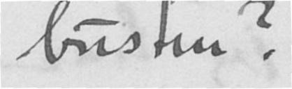busten?
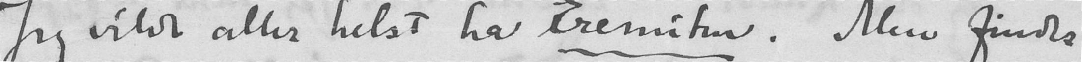Jeg vilde aller helst ha "Eremiten". Men finder
